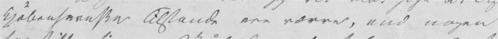kjøbenhavnske Tilstande ere værre, end nogen
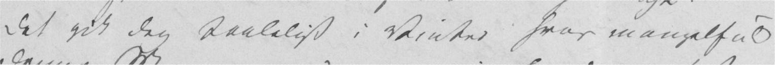Det gik dog taaleligt i Vinter hvor mangelfuld
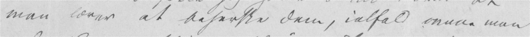man lærer at beherske dem, ialfald maae man
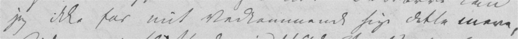jeg ikke for mit Vedkommende sige dette mere,
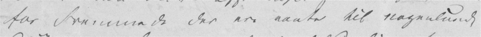for Fremmede der ere vante til nogenlunde
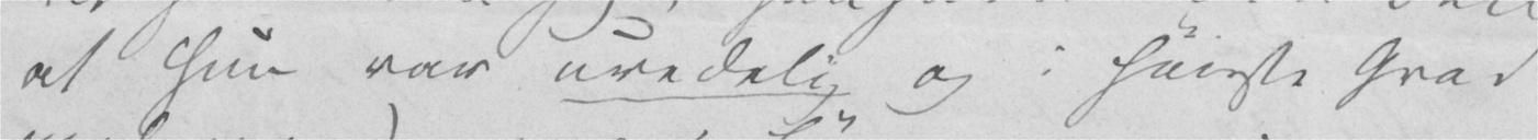at hun var uredelig og i høieste Grad
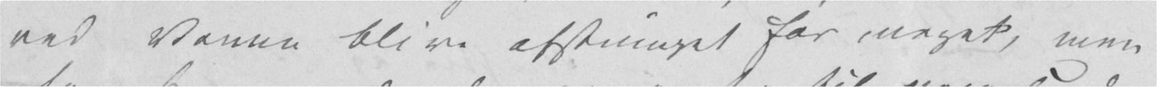ved Vanen blive afstumpet for meget, men
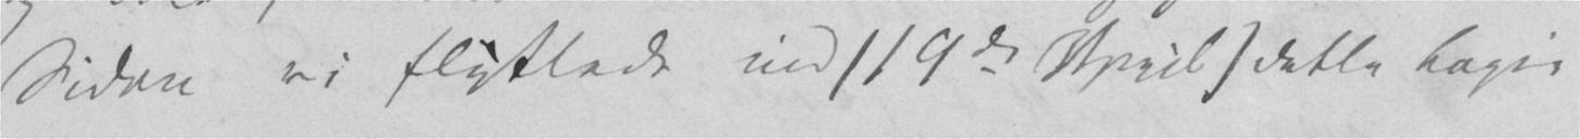siden vi flyttede ind (19de April) dette Logie
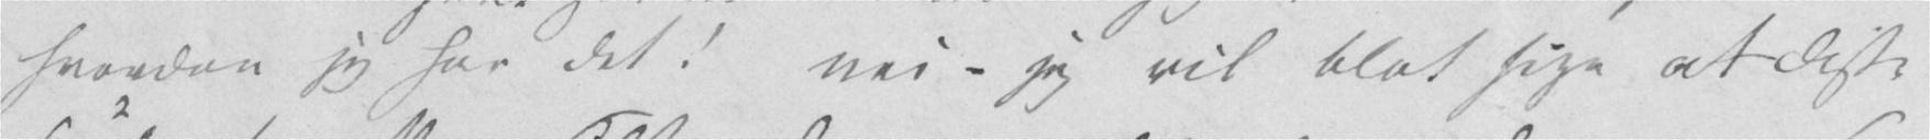hvordan jeg har det! nei – jeg vil blot sige at disse
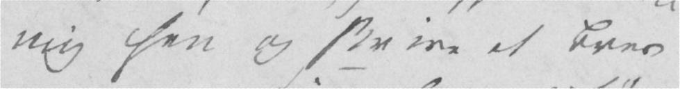mig hen og skrive et Brev
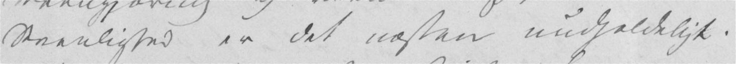Reenlighed er det næsten uudholdeligt.
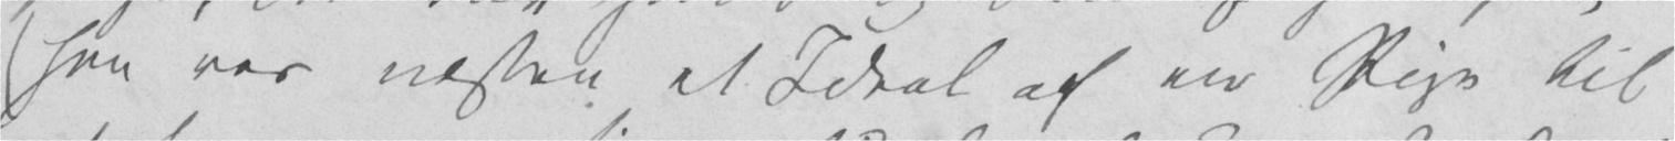(hun var næsten et Ideal af en Pige til
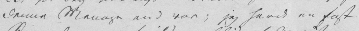denne Menage end var; jeg havde en fast
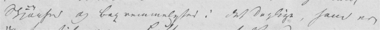Skjønhed og Beqvemmelighed i det Daglige, som ere
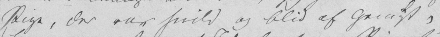Pige, der var snild og blid af Gemyt,
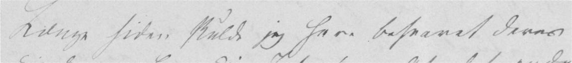Længe siden skulde jeg have besvaret Deres
